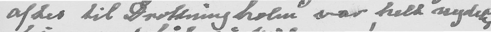aftes til Drottningholm var helt nydelig
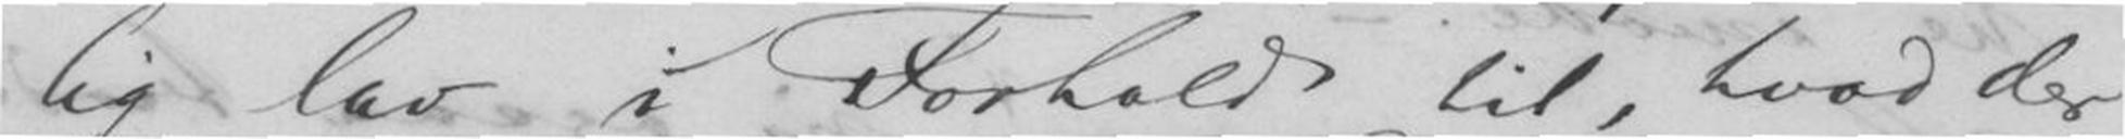lig lav i Forhold til, hvad der
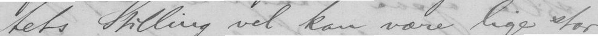tets Stilling vel kan være lige stor
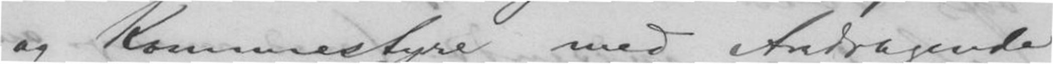og Kommunestyre med Andragende
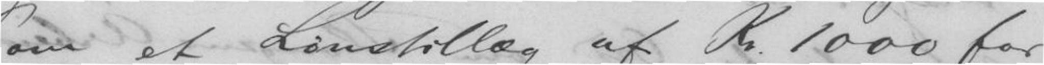om et Lønstillæg af Kr.1000 for
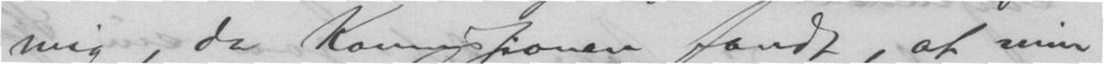mig, da Kommissionen fandt, at min
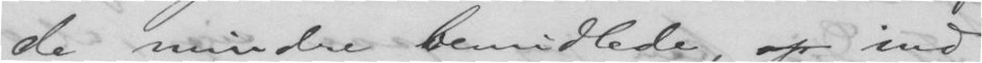de mindre bemidlede, op ind
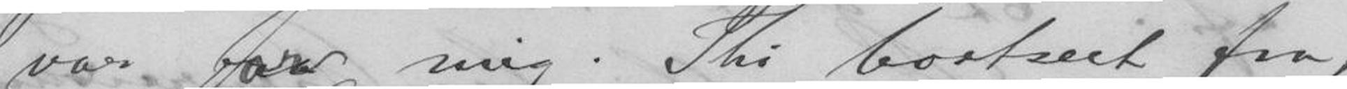var for mig. Thi bortseet fra,
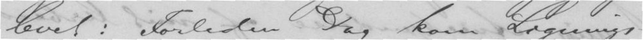levet: Forleden Dag kom Lignings-
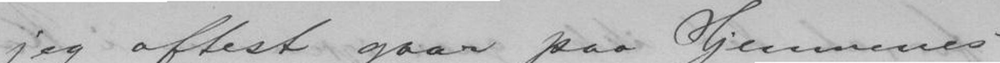jeg oftest gaar paa Hjemmenes
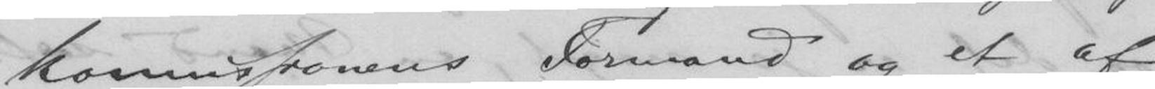kommissionens Formand og et af
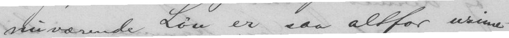nuværende Løn er saa altfor urime-
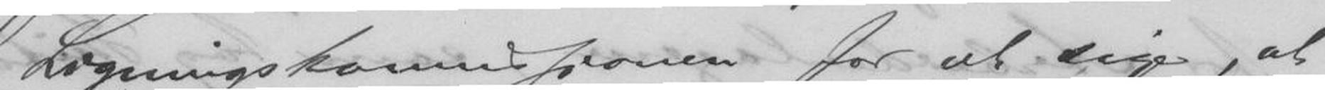Ligningskommissionen for at sige, at
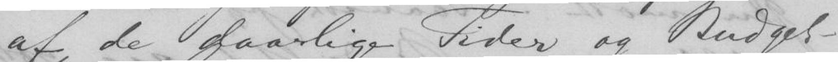af de daarlige Tider og Budget-
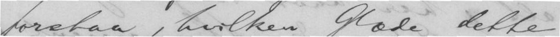forstaa, hvilken Glæde dette
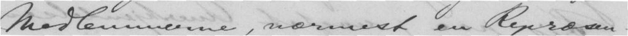Medlemmerne, nærmest en Repræsen-
